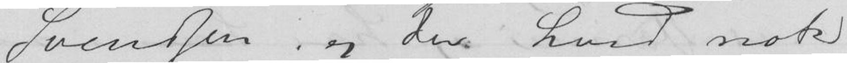Svendsen og Du hved nok
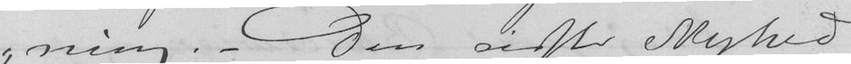ning. - Den sidste Nyhed
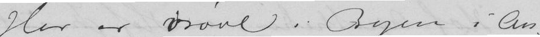Her er vrøvl i Byen i An-
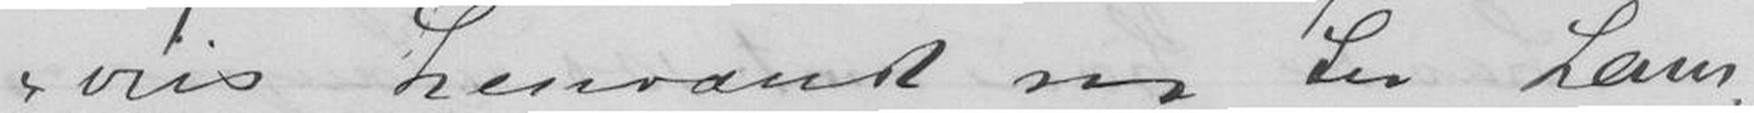vis henvændt sig til Lam-
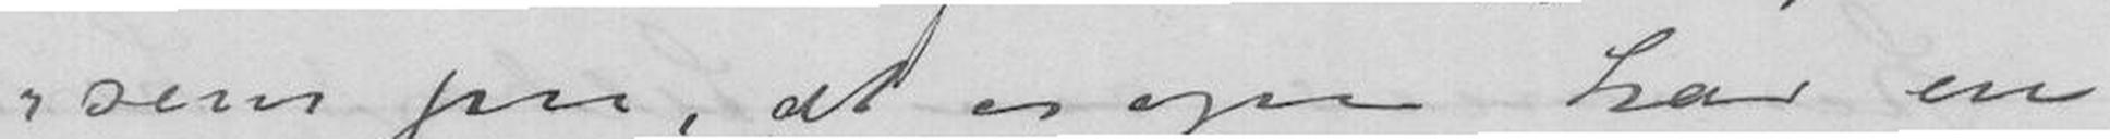som paa, at vi igen har en
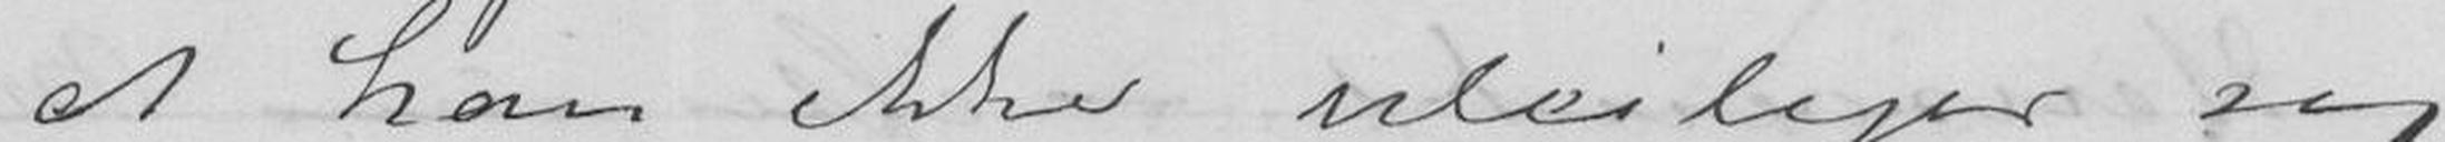at han ikke uleiliger sig
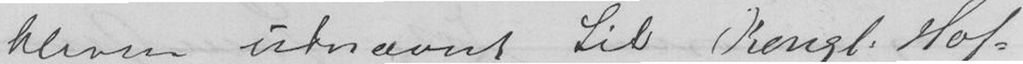bleven udnævnt til Kongl. Hof-
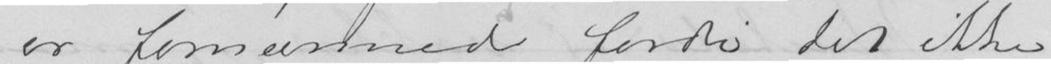er fornærmed fordi det ikke
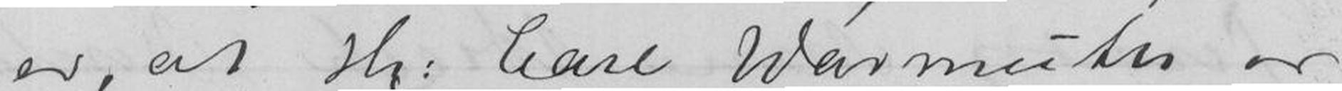er, at Hr: Carl Warmuth er
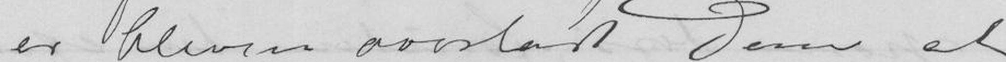er bleven overladt Dem at
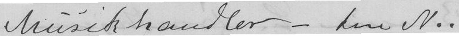Musikhandler - den N..
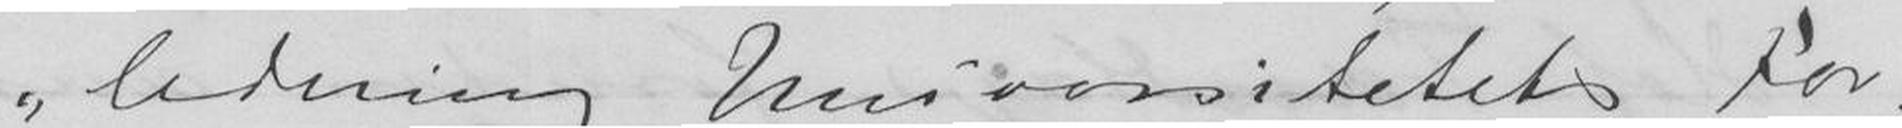ledning Universitetets For-
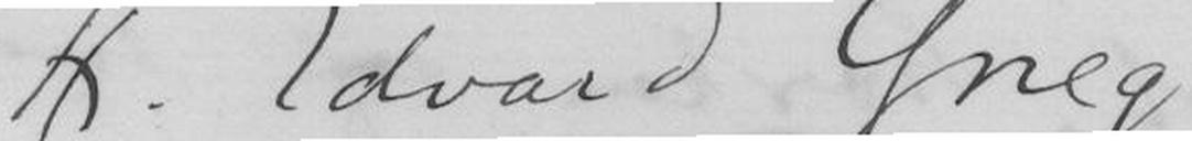H. Edvard Grieg
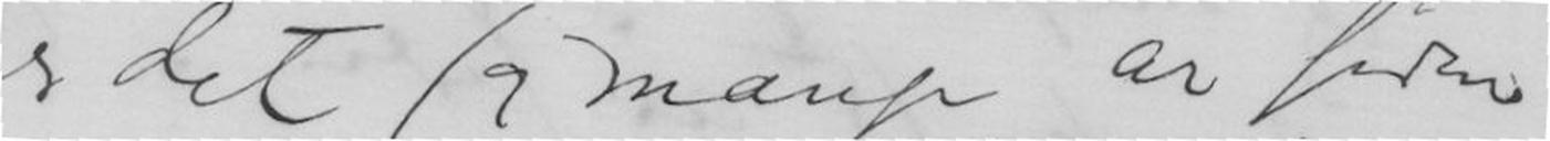er det saa mange år siden
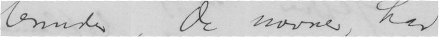lerinde, De nævne, har
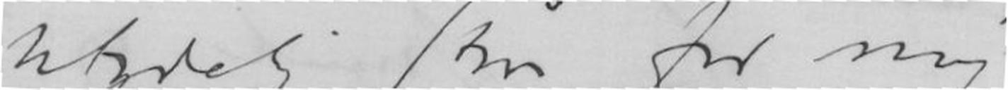utydelig står for mig.
